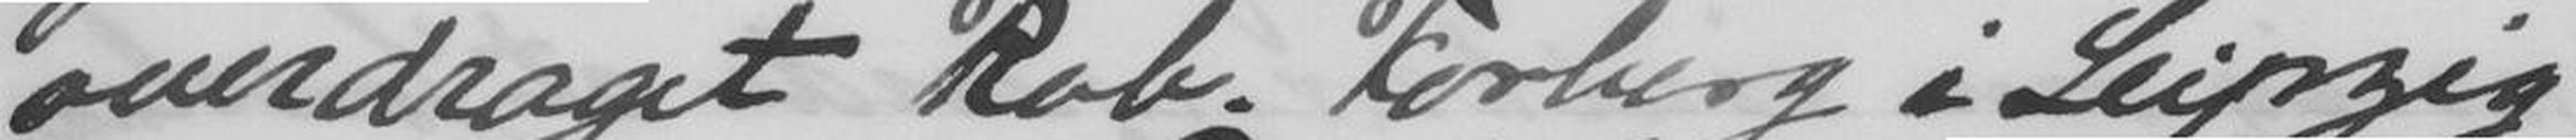overdraget Rob. Forberg i Leipzig
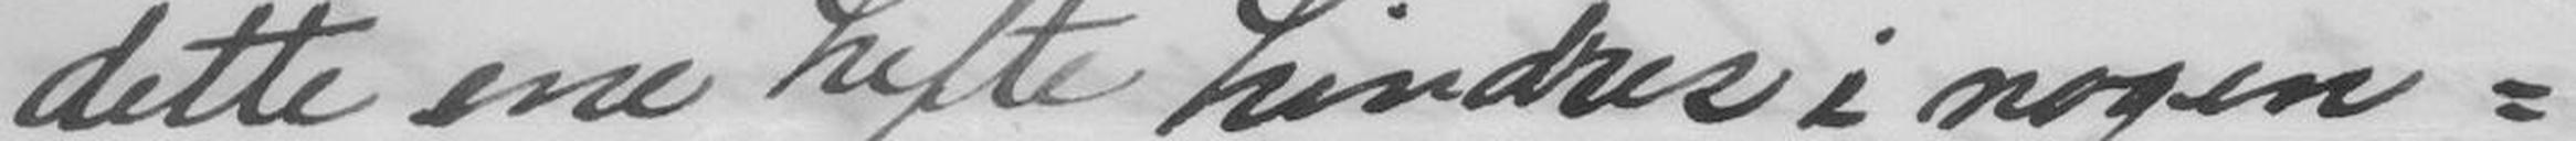dette ene hefte hindres i nogen=
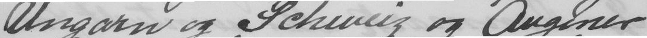Ungarn og Schweiz og Augener
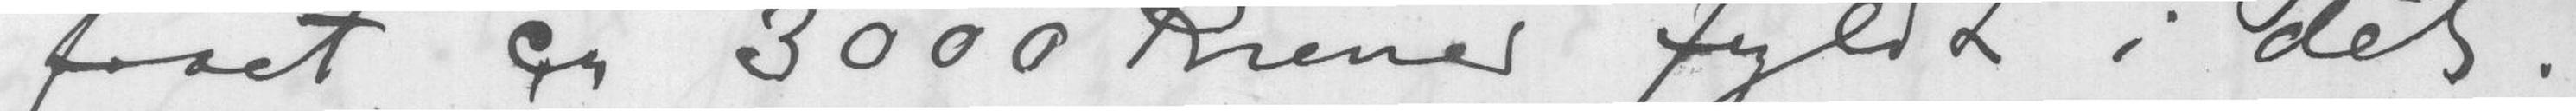faaet ca. 3000 Kroner fyldt i det
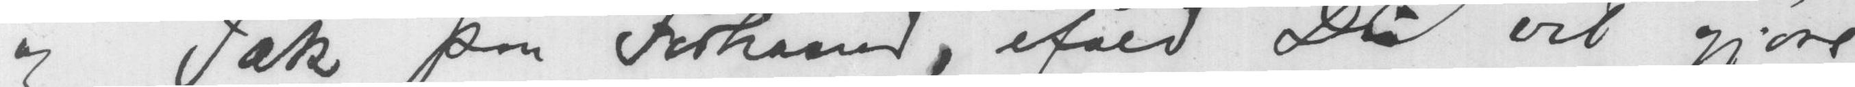og Tak paa Forhaand, ifald Du vil gjøre
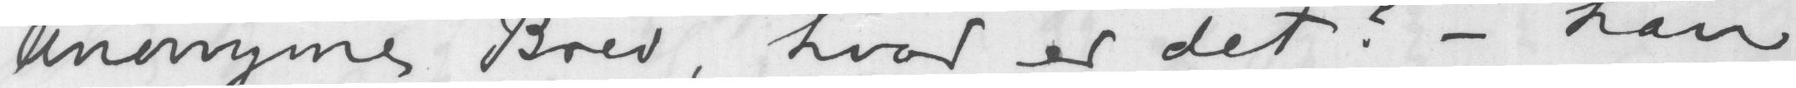anonyme Brev, hvor er det? - han
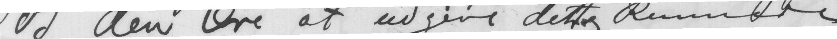os den Ære at udgive dette og kunne de
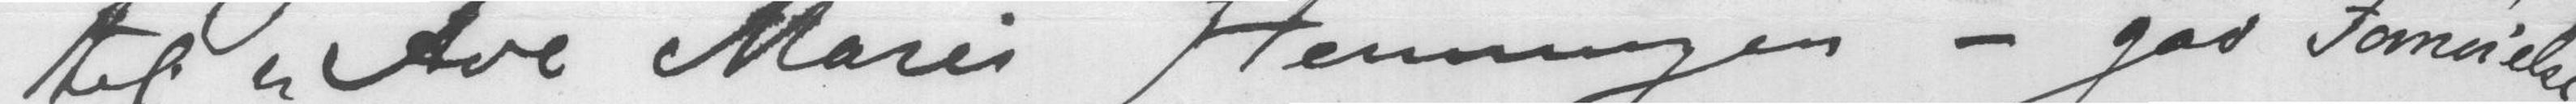til Ave Marie Stemningen- god Fornøielse,
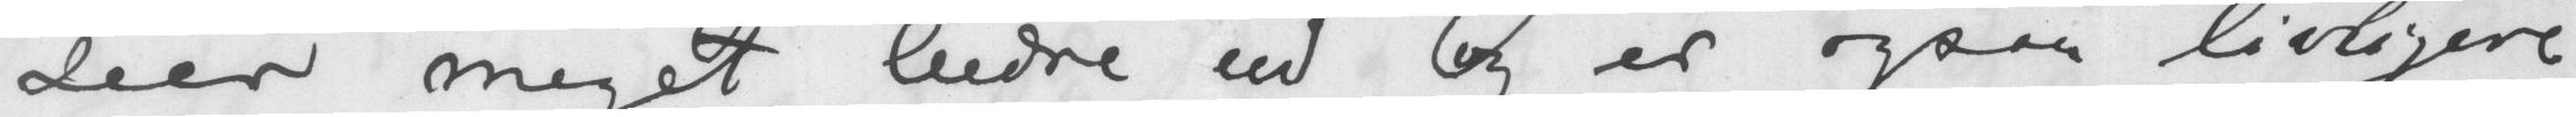seer meget bedre ud og er ogsaa livligere
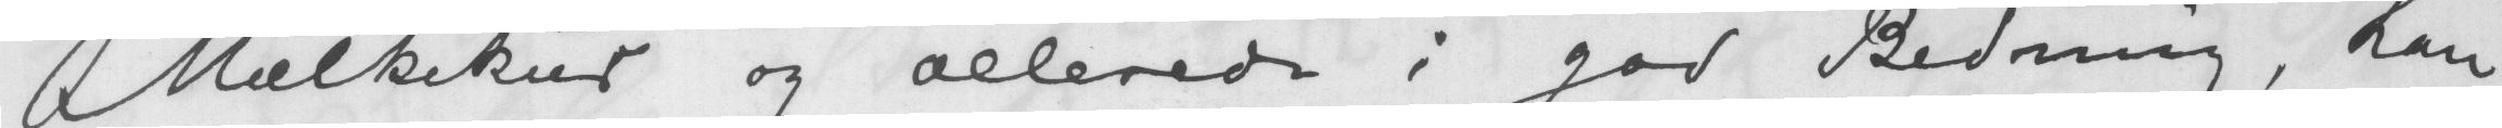Mælkekur og allerede i god Bedring, han
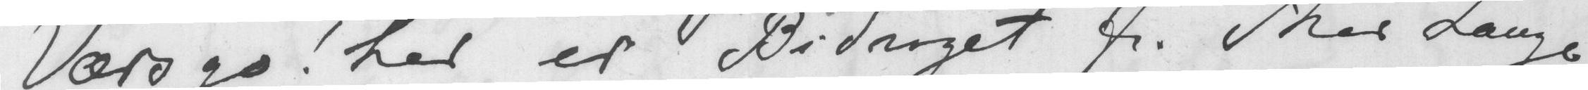Værsgo! her er Bidraget fra Thor Lange
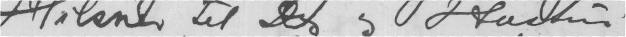Hilsener til Deg og Hustru.
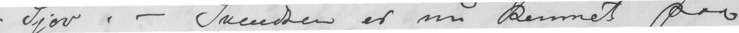- Sjov? - Svendsen er nu kommet paa
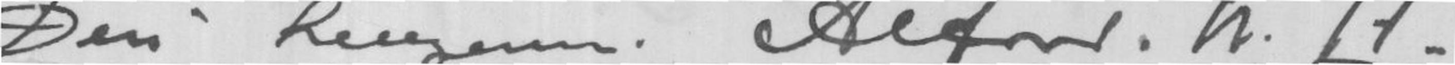Din hengivne Alfred. h II.
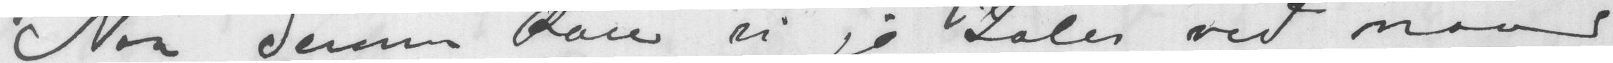Naa derom kan vi jo tales ved naar
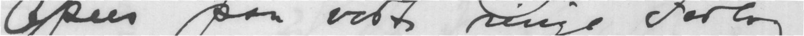opus paa vort ringe Forlag.
